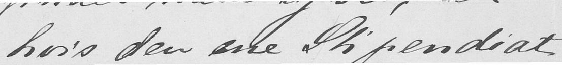hvis den ene Stipendiat
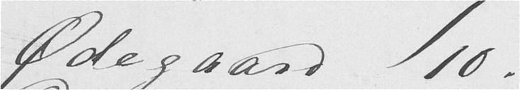Ødegaard  10.
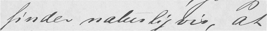finder naturligvis, at
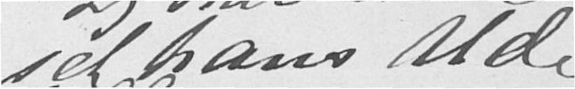set hans Ud-
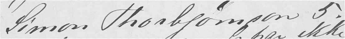Simon Thorbjørnson 5.
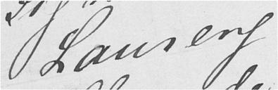Laureng 8
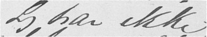Jeg har ikke
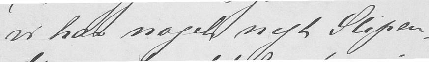vi har noget nyt Stipen-
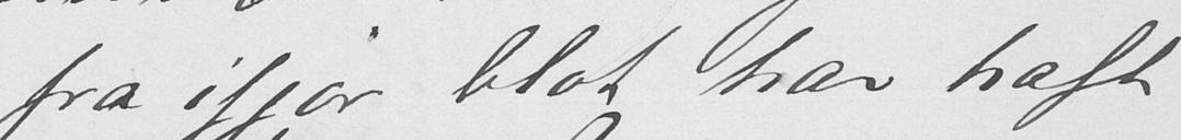fra ifjor blot har haft
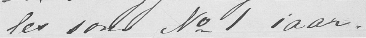les som No 1 iaar.
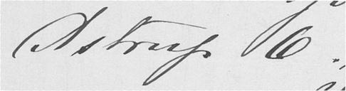Astrup 6.
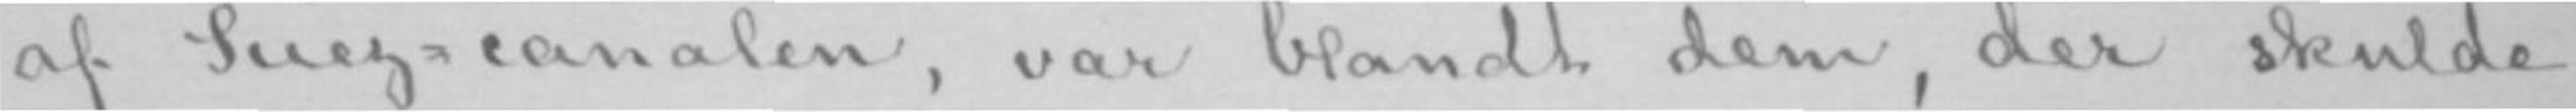af Suez-canalen, var blandt dem, der skulde
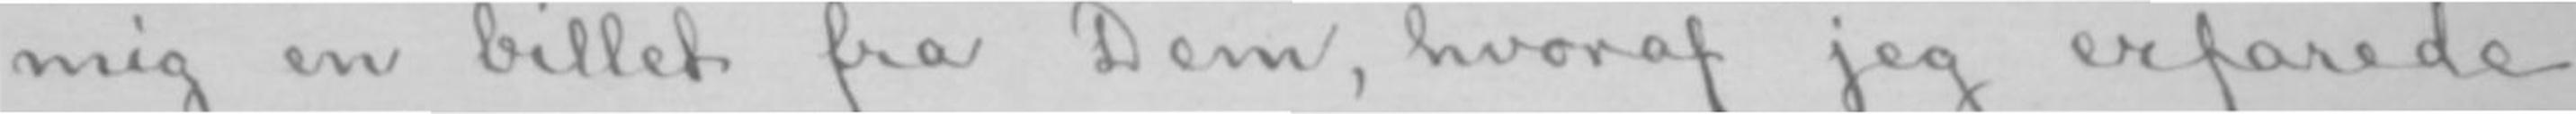mig en billet fra Dem, hvoraf jeg erfarede
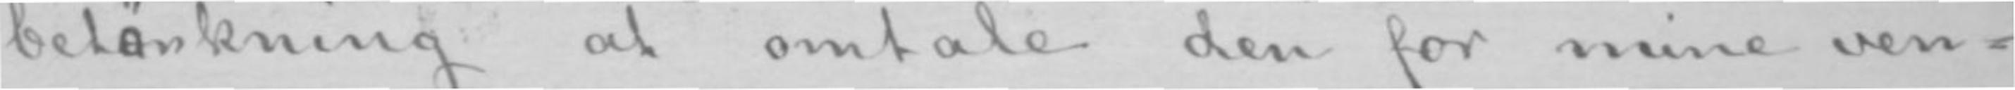betänkning at omtale den for mine ven-
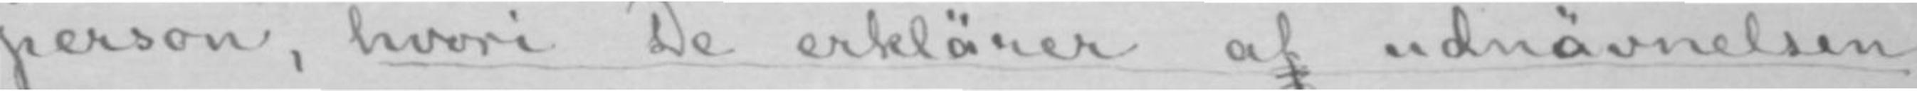person, hvori De erklärer ataf udnävnelsen
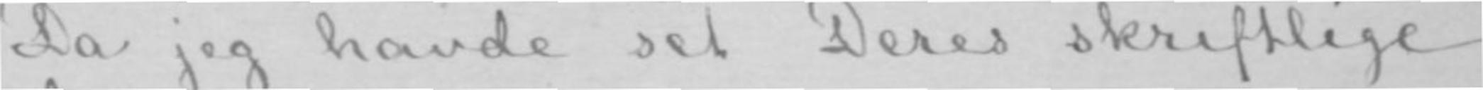Da jeg havde set Deres skriftlige
